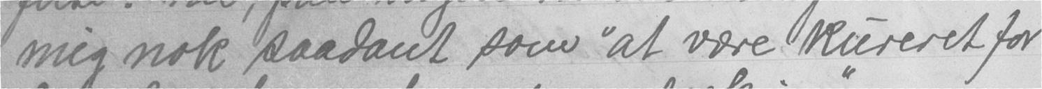mig nok saadant som "at være kureret for
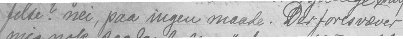felse? nei, paa ingen maade. Der foresvæver
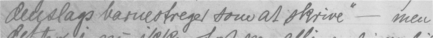denslags barnestreger som at skrive" - men
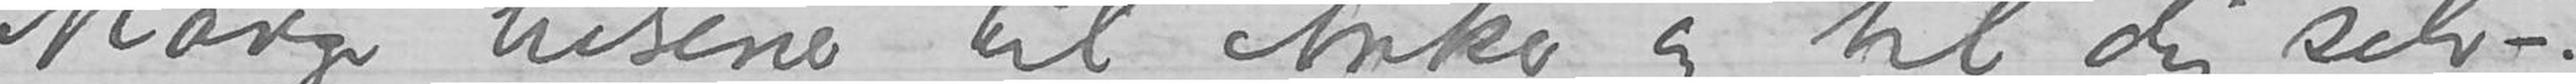Mange hilsener til Anker og til dig selv-.
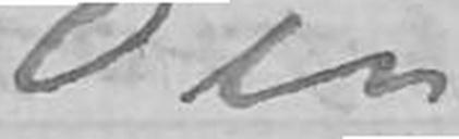Din
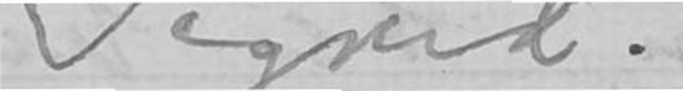Sigrid.
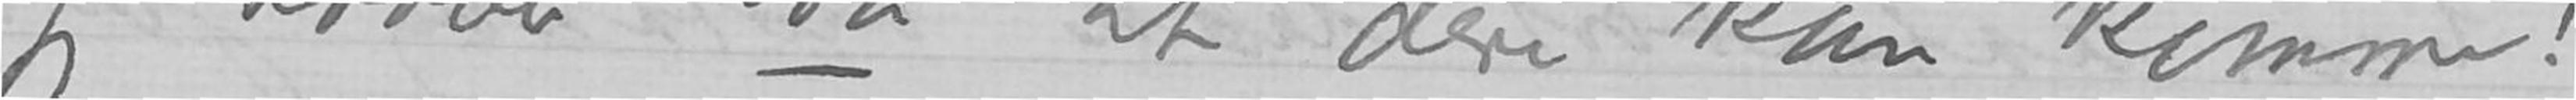Jeg haaber saa at dere kan komme!
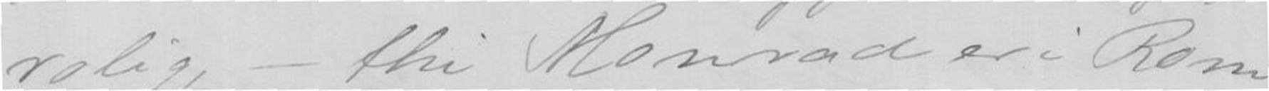rolig, - thi Monrad er i Rom
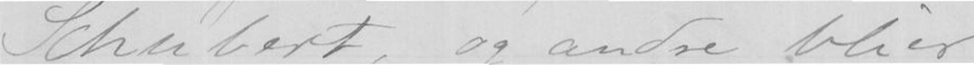Schubert, og andre blier
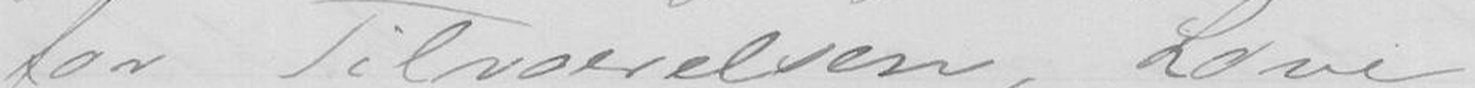for Tilværelsen, Love
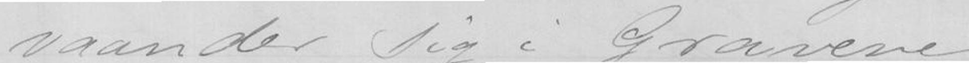vaander sig i Graverne,
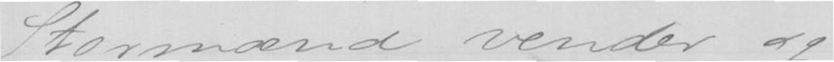Stormænd vender og
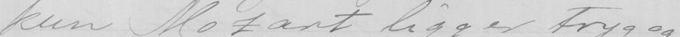kun Mozart ligger tryg og
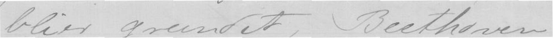blier grundet, Beethoven
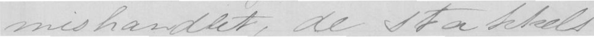mishandlet, de stakkels
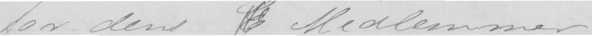for dens Medlemmer
